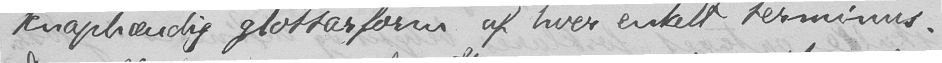knaphændig glossarform af hver enkelt terminus.
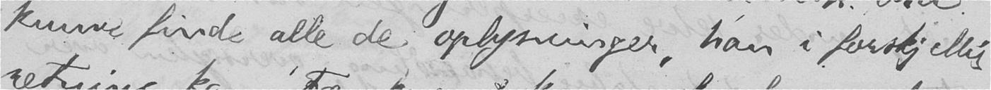kunne finde alle de oplysninger, han i forskjellig
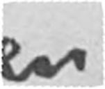en
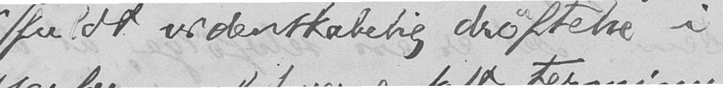fuldt videnskabelig drøftelse i
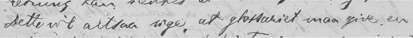Dette vil altsaa sige, at glossariet maa give en
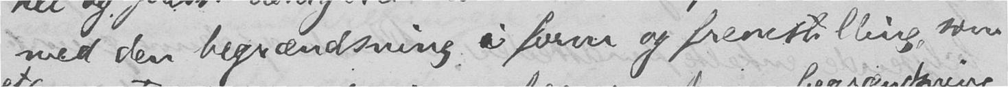med den begrændsning i form og fremstilling, som
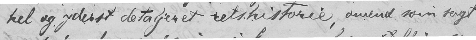hel og yderst detaljeret retshistorie, omend som sagt
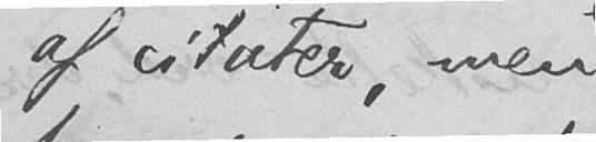af citater, men
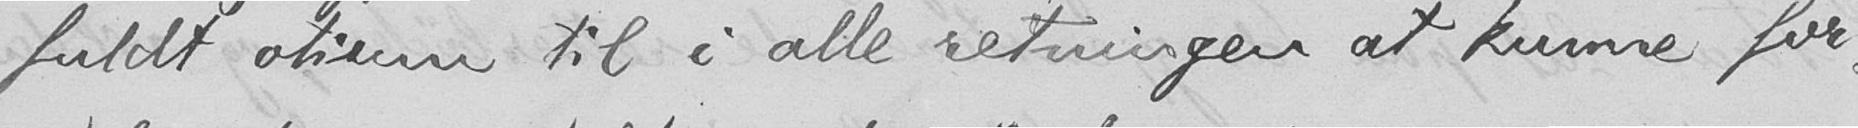fuldt otium til i alle retninger at kunne for-
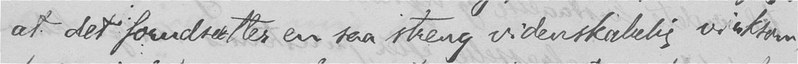at det forudsætter en saa streng videnskabelig virksom-
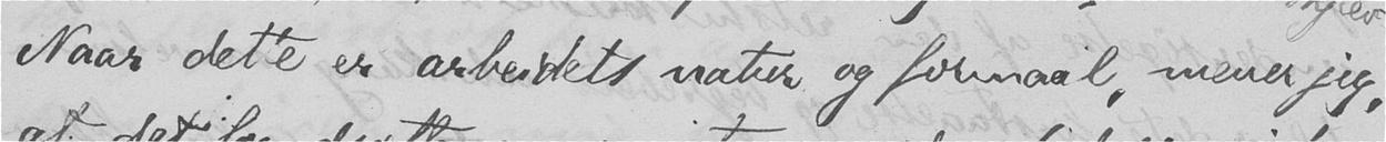Naar dette er arbeidets natur og formaal, mener jeg,
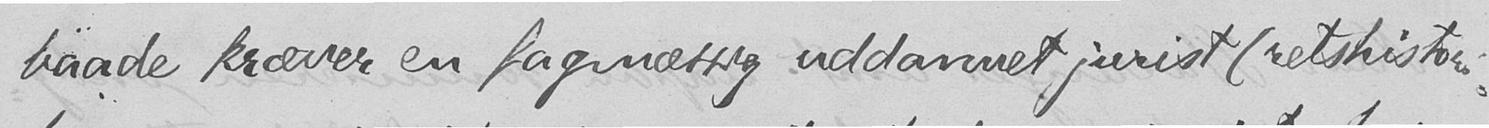baade kræver en fagmæssig uddannet jurist (retshistori-
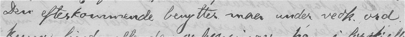Den efterkommende benytter maa under vedk. ord
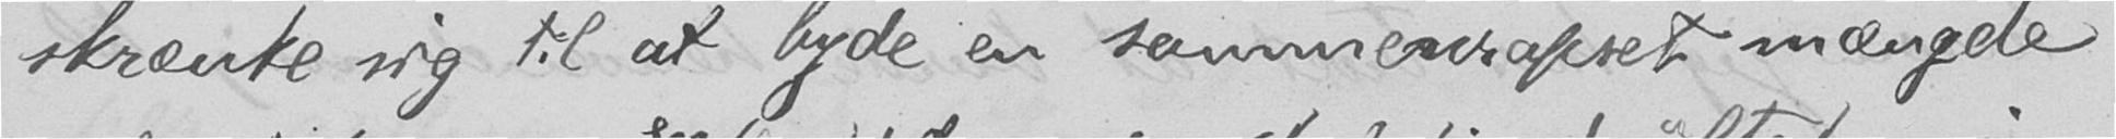skrænke sig til at byde en sammenrafset mængde
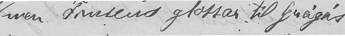men Finsens glossar til Grágás
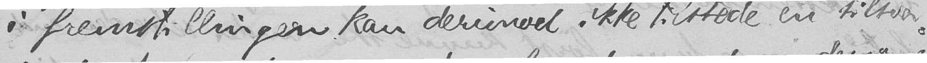i fremstillingen kan derimod ikke tilstede en tilsva-
